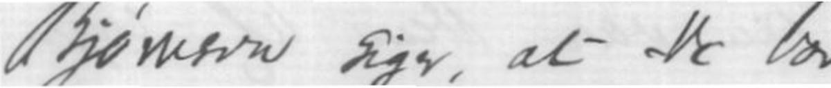Bjørnson Siger, at De bør
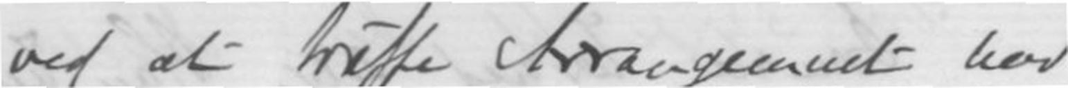ved at træffe Arrange med
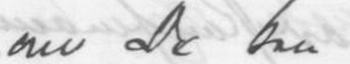om de har
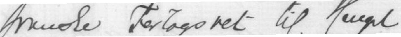fremste Forlagsret til Heugel
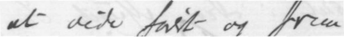at vide først og frem
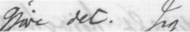gjøre det. Jeg
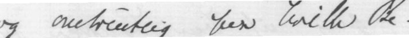og omtrentlig den hvilke Be-
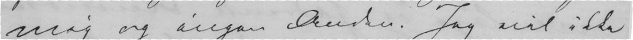mig og ingen Anden. Jeg vil ikke
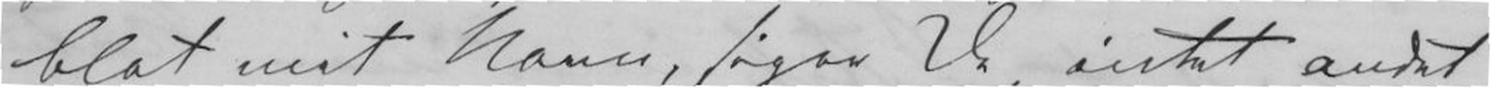blot mit Navn, siger De, intet andet
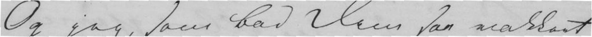Og jeg, som bad Dem saa vakkert
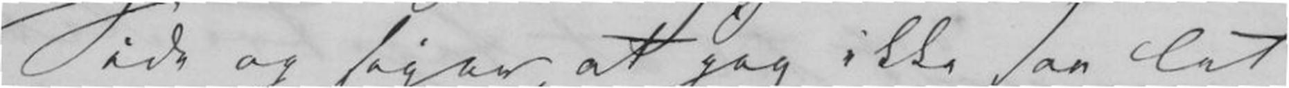Side og siger, at jeg ikke saa det
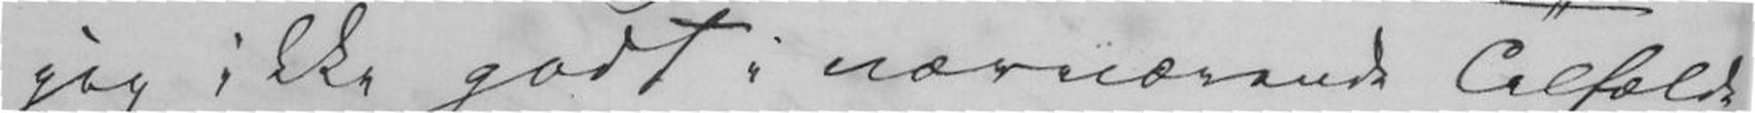jeg ikke godt i nærværende Tilfælde
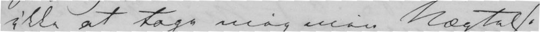ikke ar tage mig min agtets-
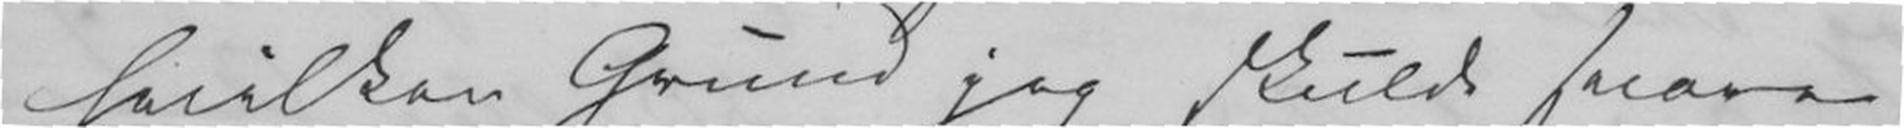hvilken Grund jeg skulde svare
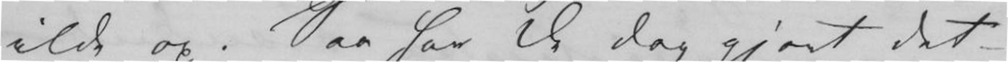ilde op. Saa har De dog gjort det
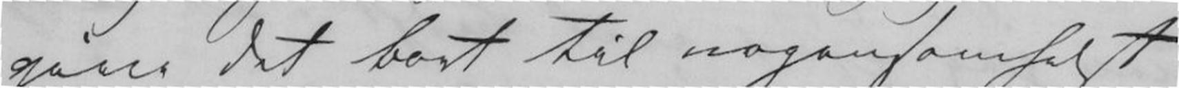give det bort til nogensomhelst
